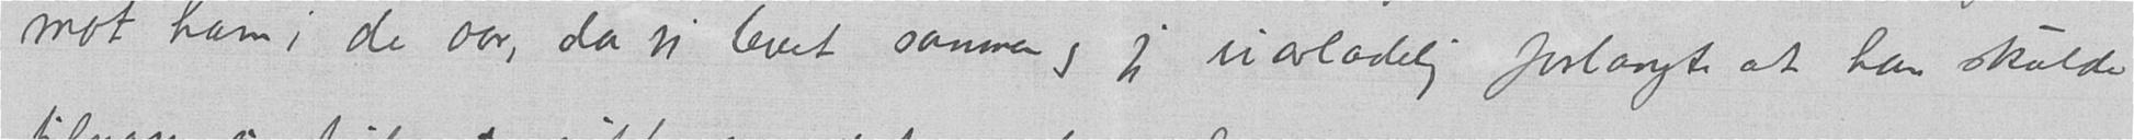mot ham i de aar, da vi levet sammen og jeg uavladelig forlangte at han skulde
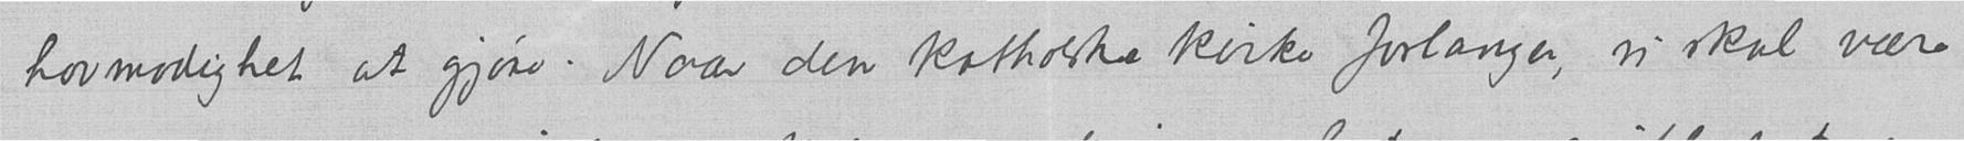hovmodighet at gjøre. Naar den katholske kirke forlanger, vi skal være
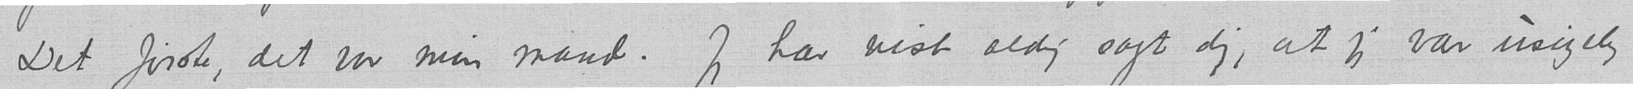Det første, det var min mand. Jeg har vist aldrig sagt dig, at jeg var usigelig
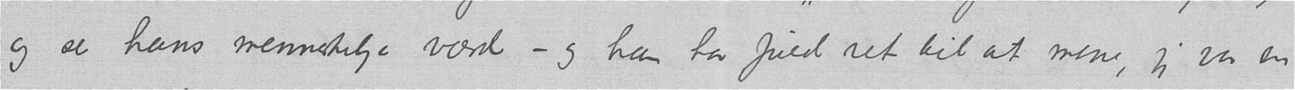og se hans menneskelige værd – og han har fuld ret til at mene, jeg var en
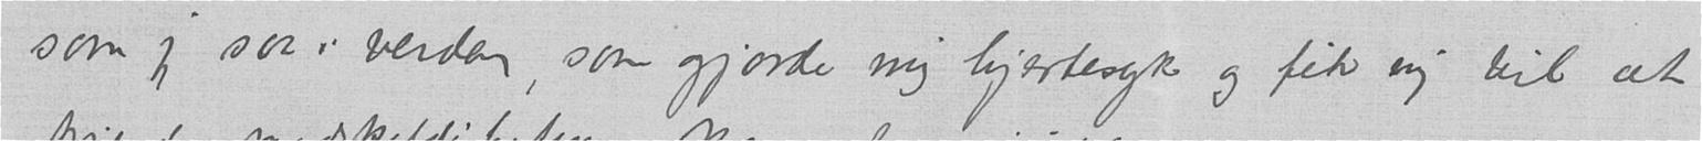som jeg saa i verden som gjorde mig hjertesyk og fik mig til at
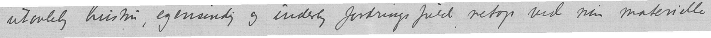utaalelig hustru, egensindig og inderlig fordringsfuld, netop ved min materielle
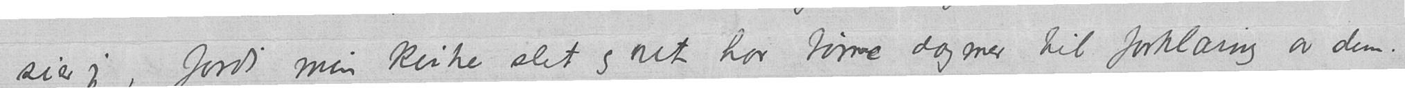sier jeg, fordi min kirke slet og ret har tørre dogmer til forklaring av dem.
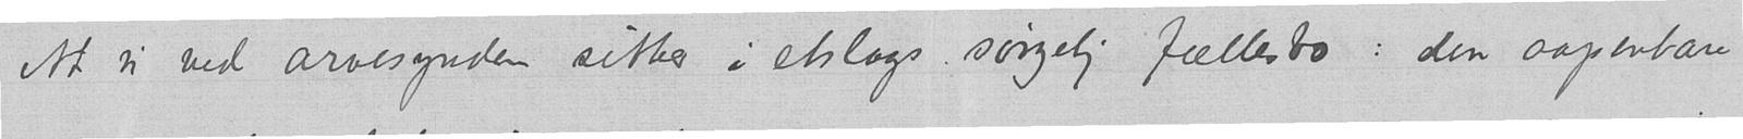At vi ved arvesynden sitter i etslags sørgelig fællesbo: den aapenbare
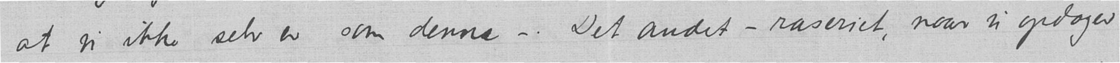at vi ikke selv er som denne –. Det andet – raseriet, naar vi opdager
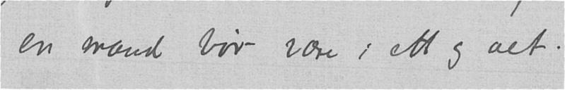en mand bør være i ett og alt.
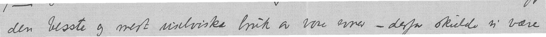den besste og mest uselviske bruk av vore evner – derfor skulde vi være
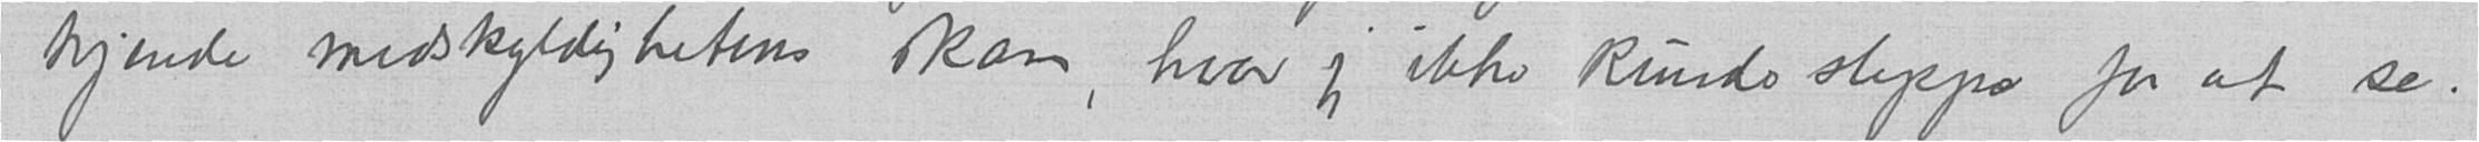kjende medskyldighetens skam, hvor jeg ikke kunde slippe for at se.
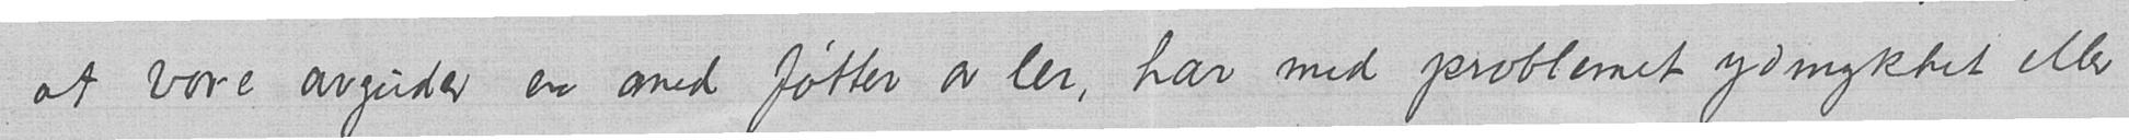at vore avguder er med føtter av ler, har med problemet ydmykhet eller
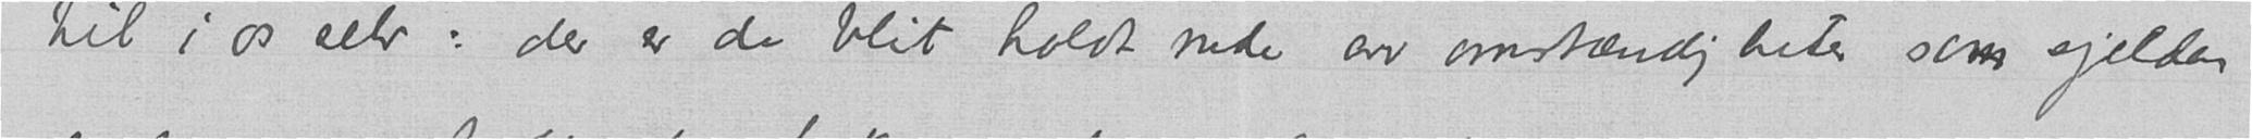til i os selv: der er de blit holdt nede av omstændigheter som sjelden
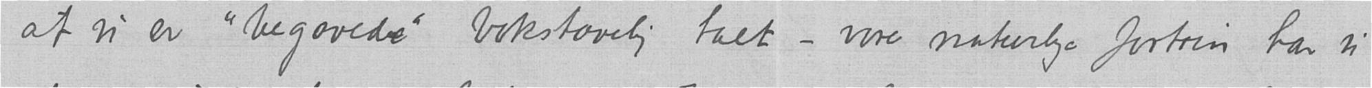at vi er "begavede" bokstavelig talt – vore naturlige fortrin har vi
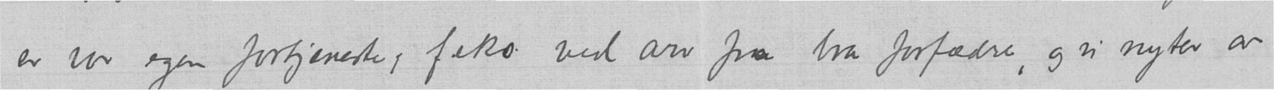er vor egen fortjeneste, feks. ved arv fra bra forfædre, og vi nyter av
